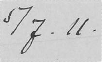5/7.11.
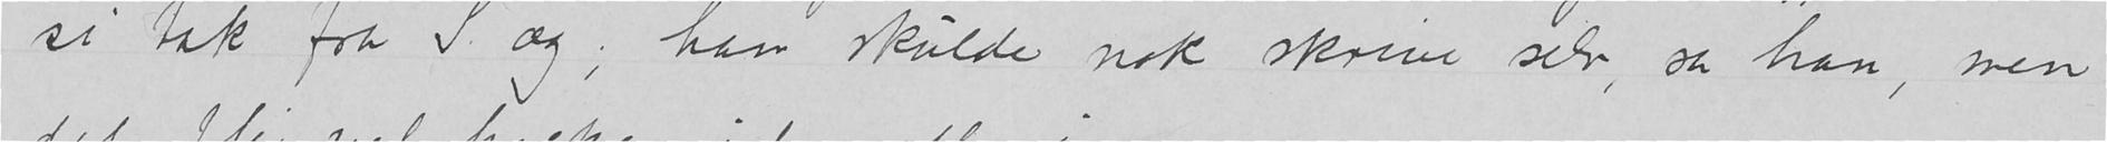si tak fra S. og, han skulde nok skrive selv, sa han, men
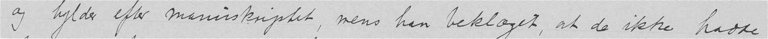og hylder efter manuskriptet, mens han beklaget, at de ikke hadde
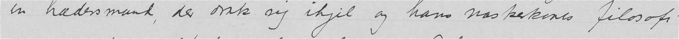en hædersmand, der drak sig ihjel og hans vaskekones filosofi
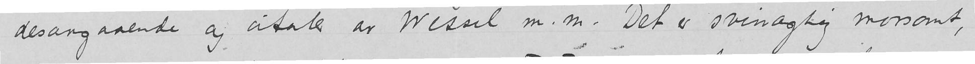desangaaende og utaler av Wessel mm. Det er svinagtig morsomt,
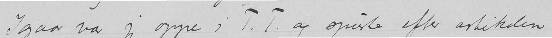Igaar var jeg oppe i T.T. og spurte efter artikelen
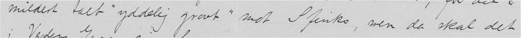mildest talt «yddelig grovt» mot Sfinks, men da skal det
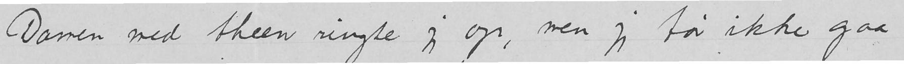Damen med theen ringte jeg op, men jeg får ikke gaa
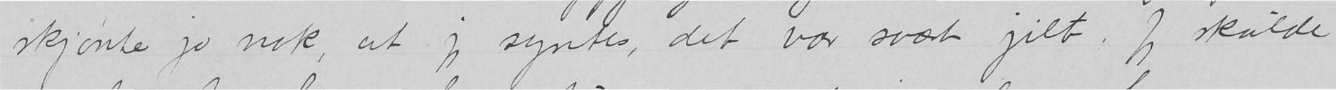skjønte jo nok, at jeg syntes det var svært jilt.  Jeg skulde
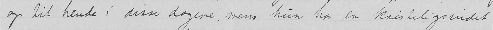op til hende i disse dagene, mens hun har en kristeligsindet
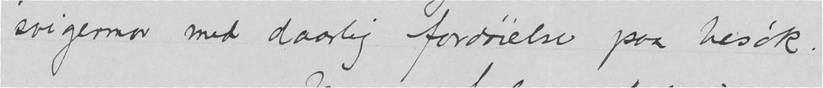svigermor med daarlig fordøielse paa besøk.
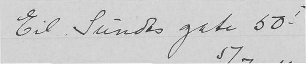Eil. Sundts gate 50
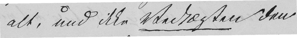alt, und ikke Vedtægten den
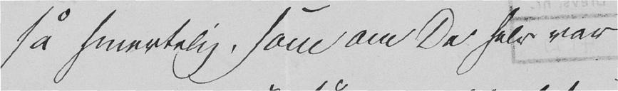så smertelig, som om De selv var
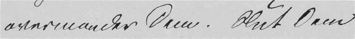overmander Dem. Slut Dem
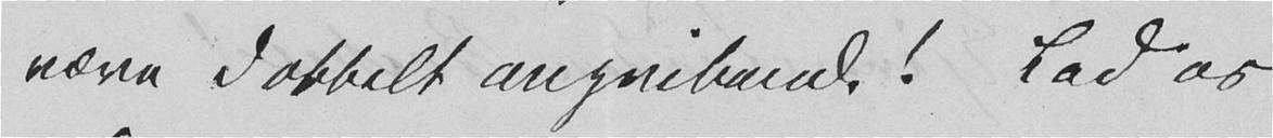være dobbelt angribende! Lad os
